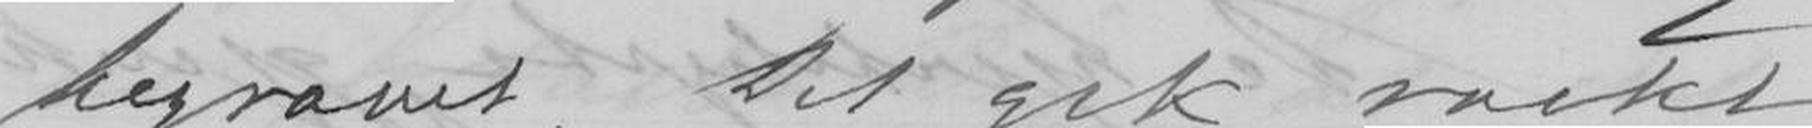begravet. Det gik raskt
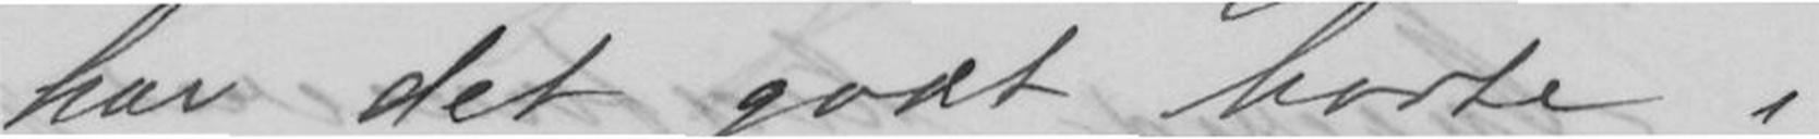har det godt borte i
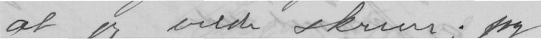at jeg vilde skrive; jeg
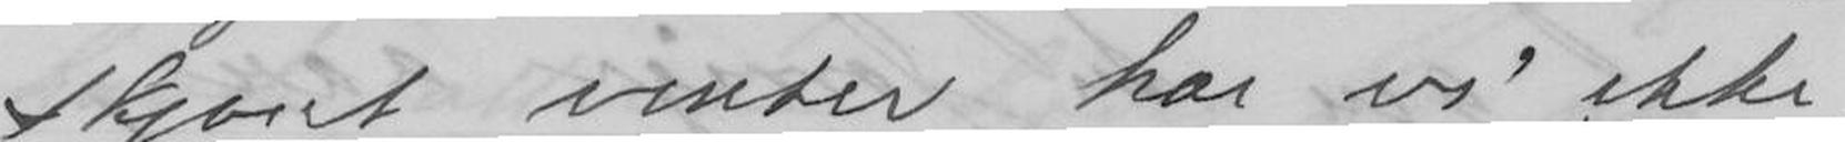skjønt vinter har vi ikke.
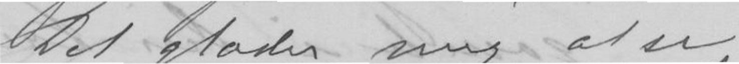Det glæder mig at se,
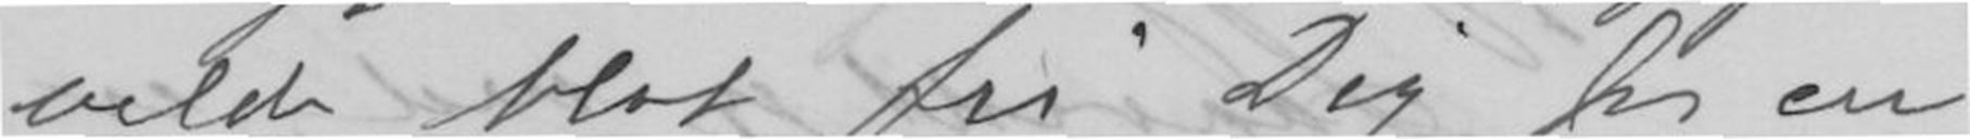vilde blot fri Dig for en
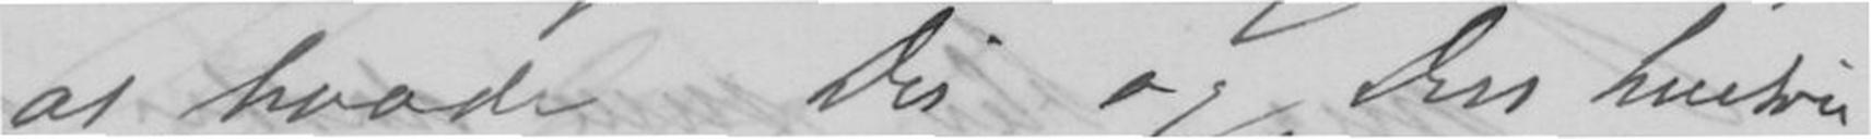at baade Du og Din hustru
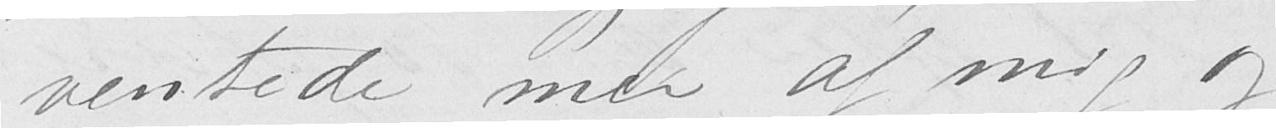ventede mer af mig og
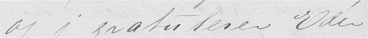og jeg gratulerer Eder
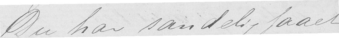Du har sandelig faaet
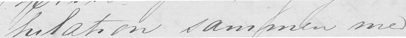tulation sammen med
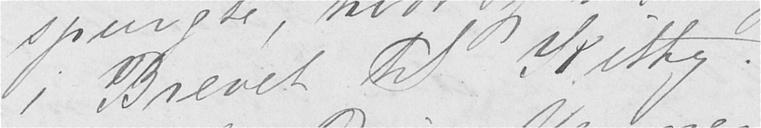i Brevet til Kitty.
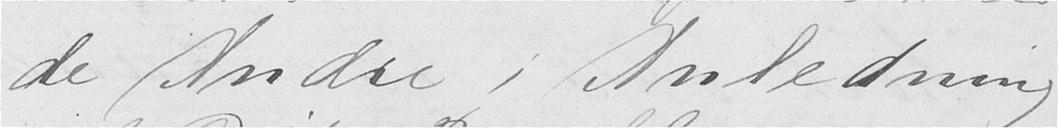de Andre i Anledning
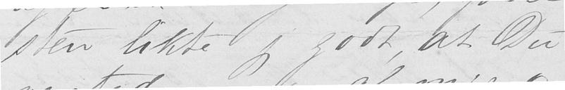sten likte jeg godt, at Du
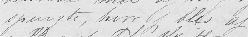spurgte, hvor jeg blev af
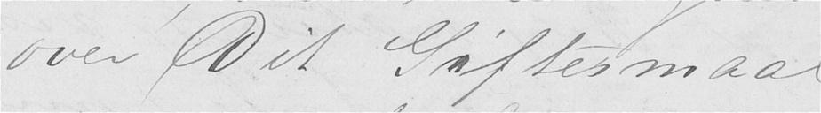over Dit Giftermaal,
